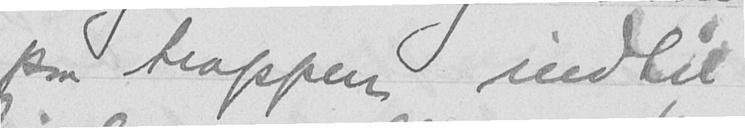paa trappen indtil
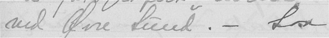ved Øvre Sund. - Saa
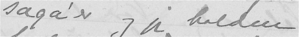saga'er og jeg holden
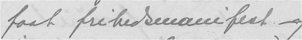faat frihedsmanifest - og
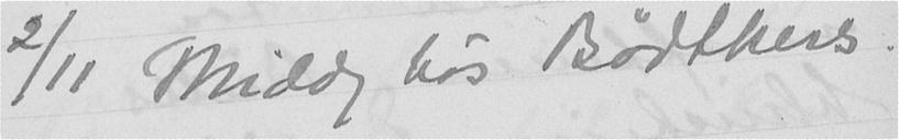2/11 Middag hos Bødtkers.
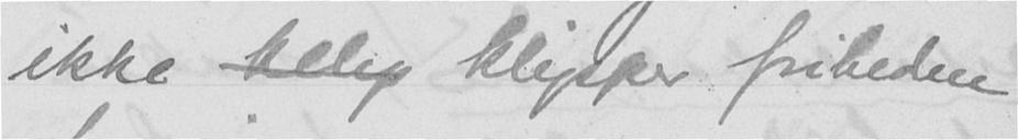ikke kllip klipper friheden
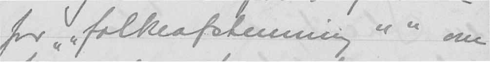for ""folkeafstemning"" om
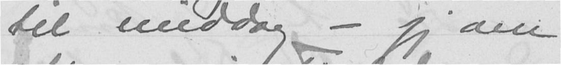til middag - jeg om
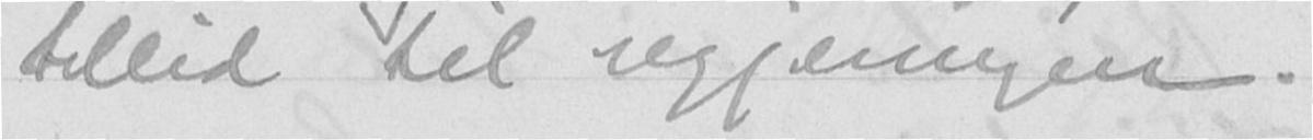tillid til regjeringen.
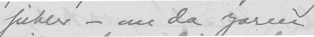jubler - om da zaren
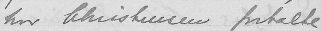hvor Christensen fortalte
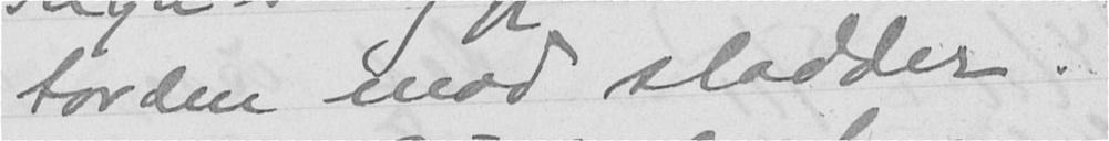torden mod sladder.
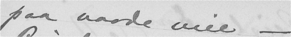paa vaade veie -
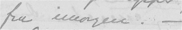fra imorgen. -
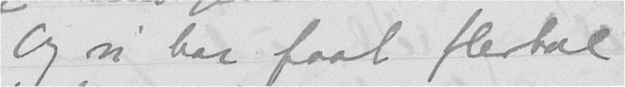Og vi har faat flertal
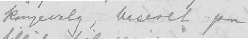kongevalg, baseret paa
